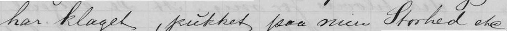har klaget, pukket paa min Storhed etc,
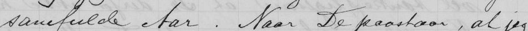samfulde Aar. Naar De paastaar, at jeg
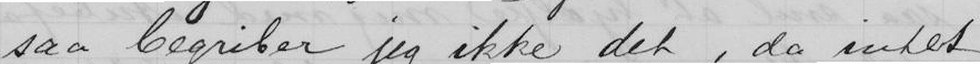saa begriber jeg ikke det, da intet
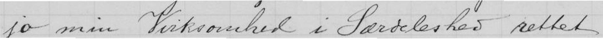jo min Virksomhed i Særdeleshed rettet
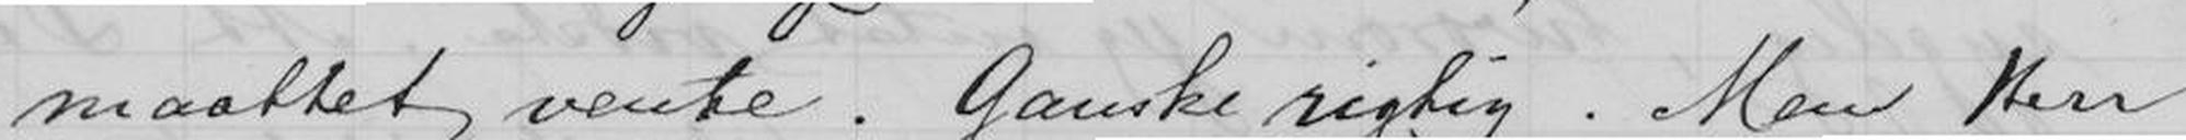maattet vente. Ganske rigtig. Men Herr
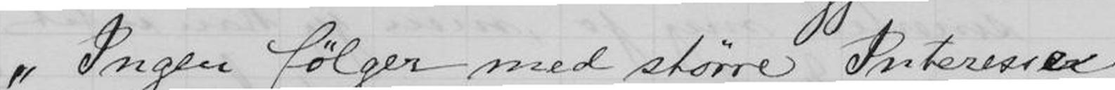"Ingen følger med større Intresse
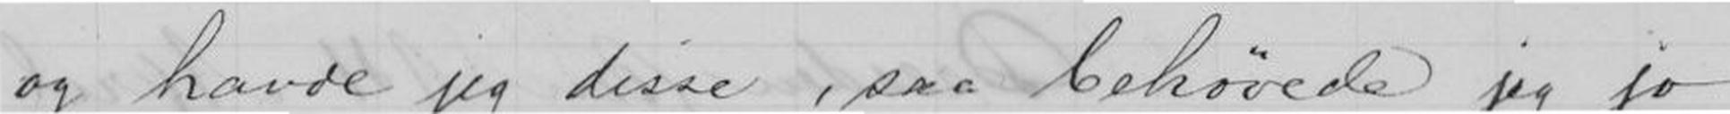og havde jeg disse, saa behøvede jeg jo
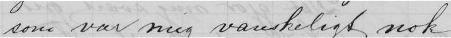som var mig vanskeligt nok.
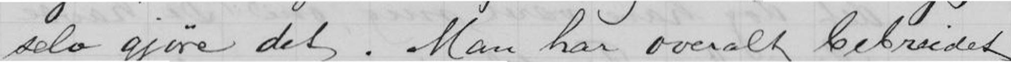selv gjøre det. Man har overalt bebreidet
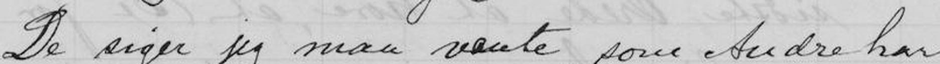De siger jeg maa vente, som Andre har
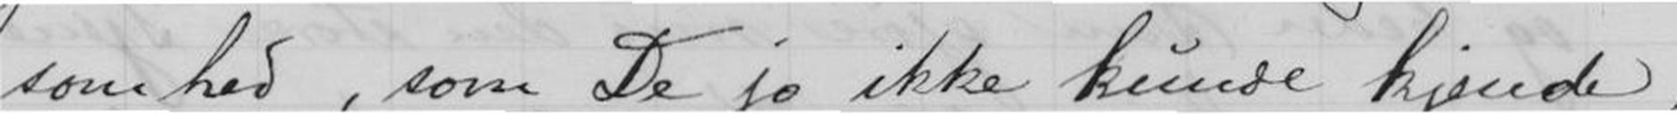somhed, som De jo ikke kunde kjende,
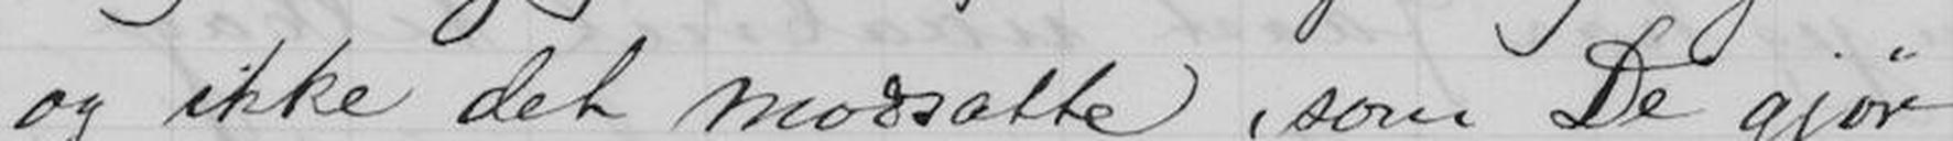og ikke det modsatte, som De gjør. -
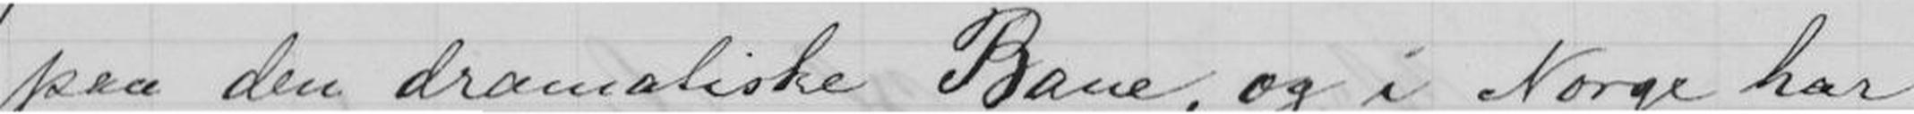paa den dramatiske Bane, og i Norge har
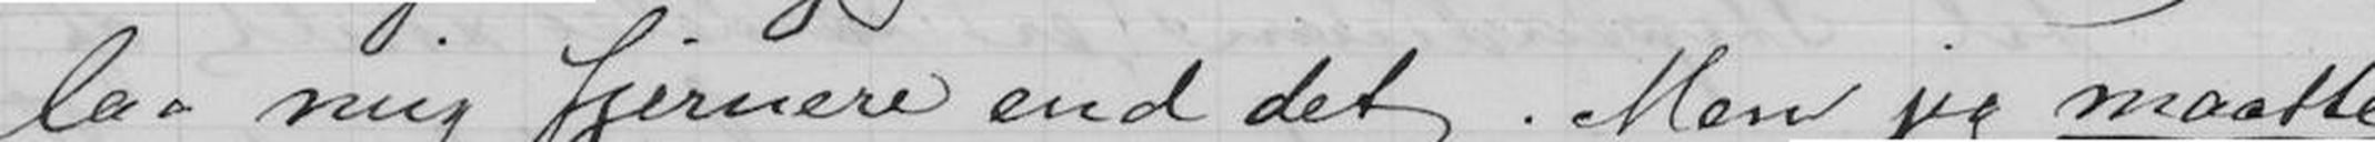laa mig fjernere end det. Men jeg maatte
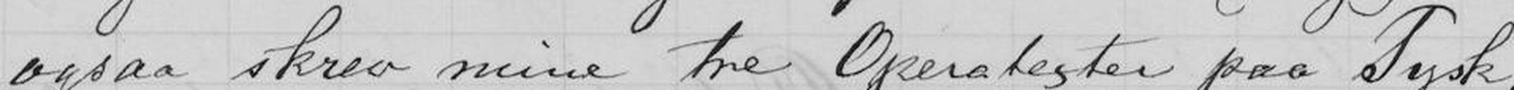ogsaa skrev mine tre Operatexter paa Tysk,
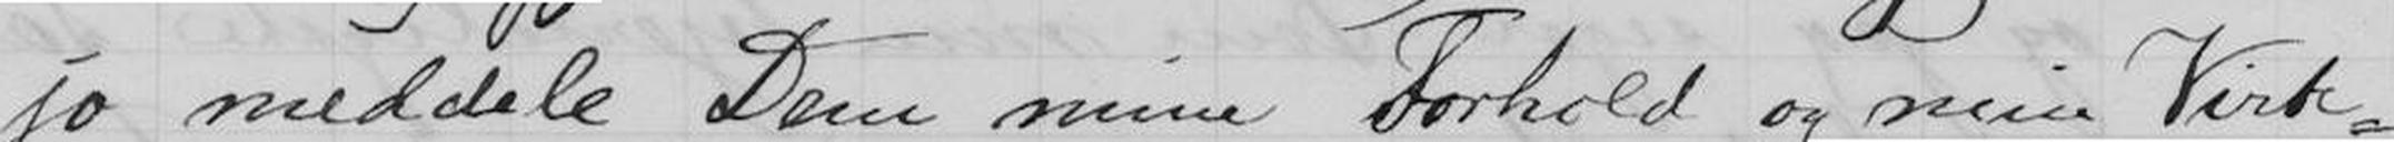jo meddele Dem mine Forhold og min Virk-
